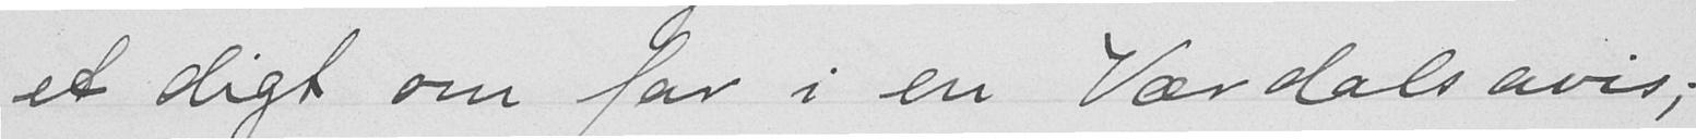et digt om far i en Værdalsavis;
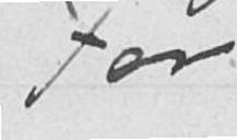For
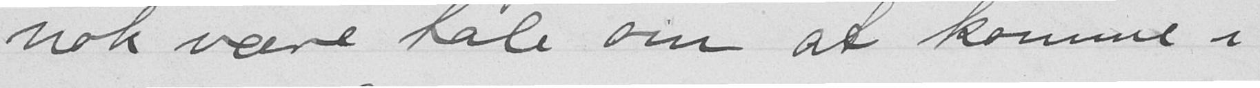nok være tale om at komme i
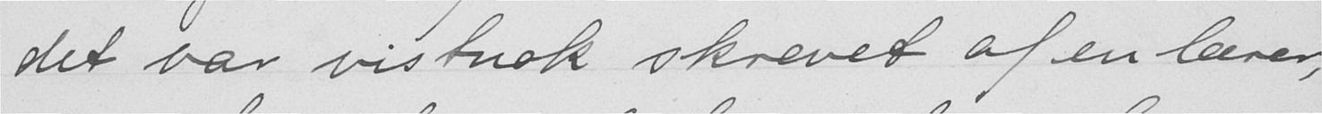det var vistnok skrevet af en lærer,
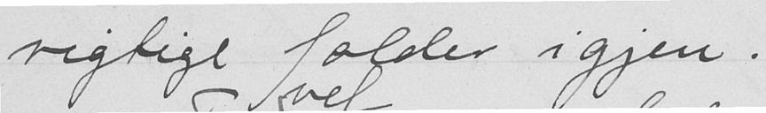rigtige folder igjen.
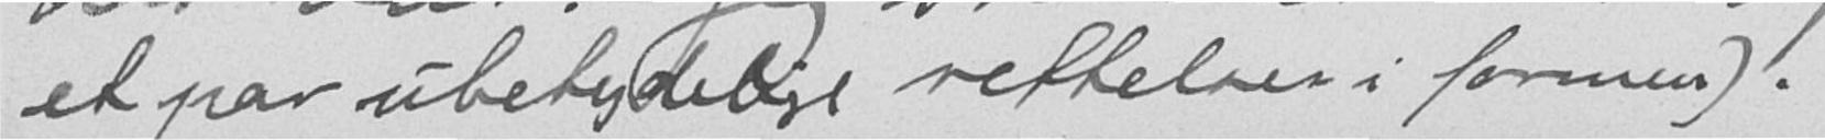et par ubetydelige rettelser i formen).
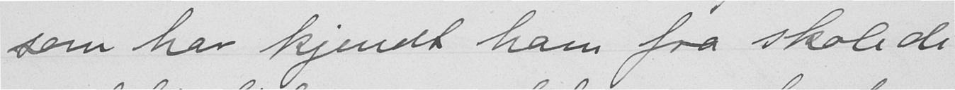som har kjendt ham fra skoledi
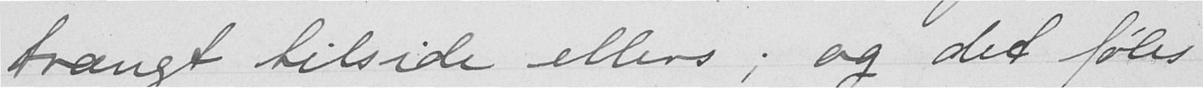trængt tilside ellers; og det føles
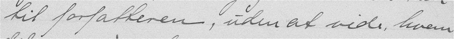til forfatteren, uden at vide, hvem
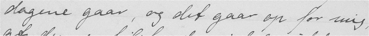dagene gaar, og det gaar op for mig,
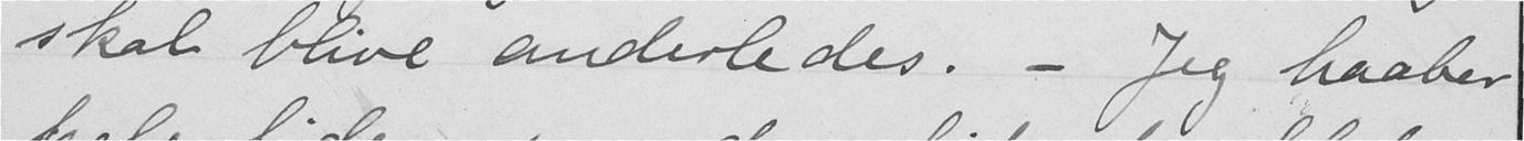skal blive anderledes. - Jeg haaber
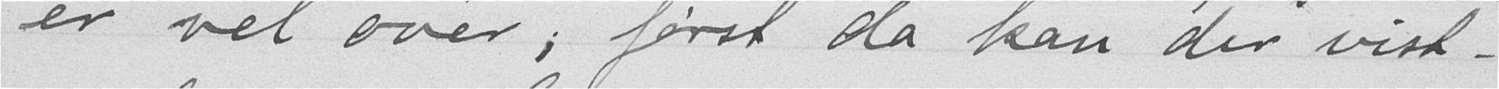er vel over; først da kan der vist-
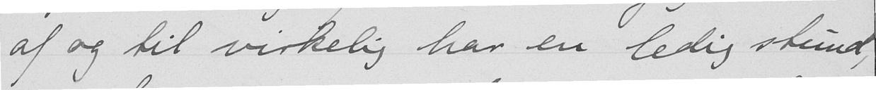af og til virkelig har en ledig stund,
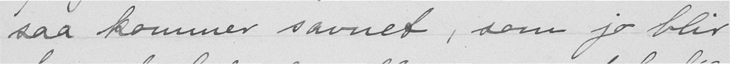saa kommer savnet, som jo blir
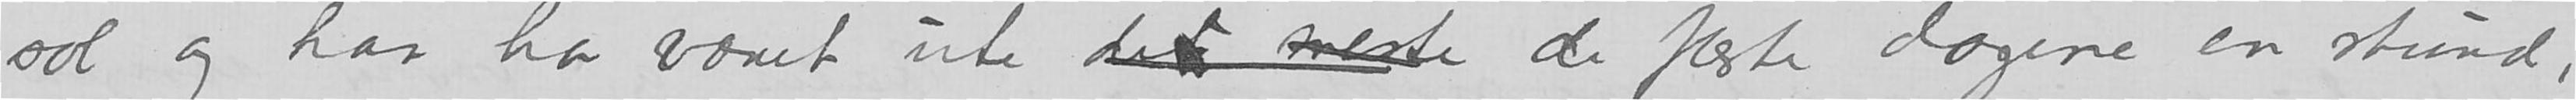sol og han har været ute det meste de fleste dagene en stund,
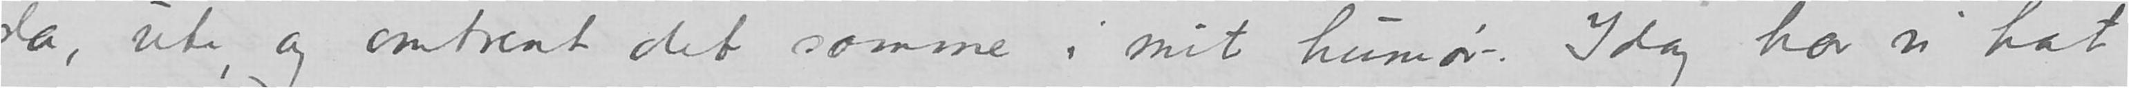da, ute, og omtrent det samme i mit humør-. Idag har vi hat
